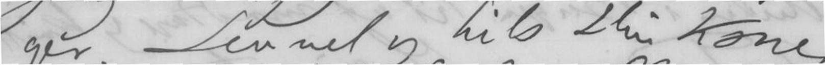ger. Lev vel og hils Din Kone,
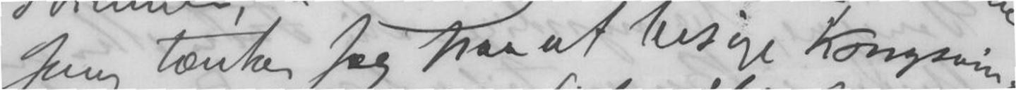Gang tænker Jeg paa at besøge Kongsvin-
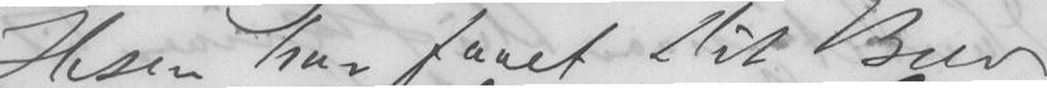Ibsen har faaet Dit Brev.
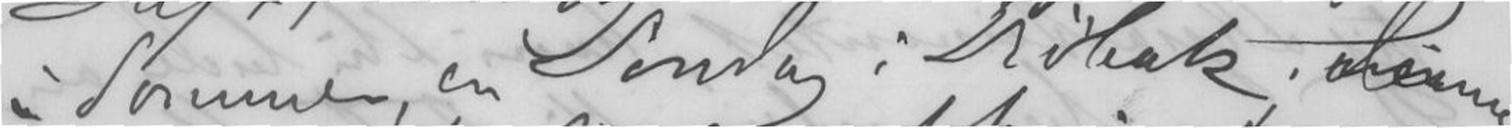i Sommer, en Søndag i Drøbak. Denne
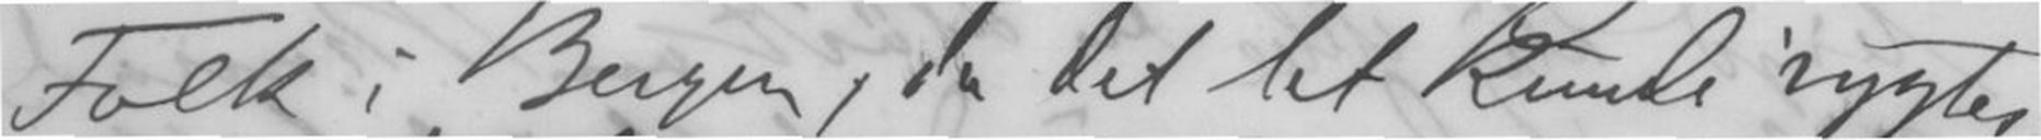Folk i Bergen, da det let kunde rygtes
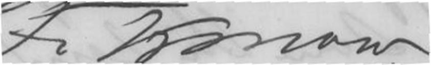Fr Konow.
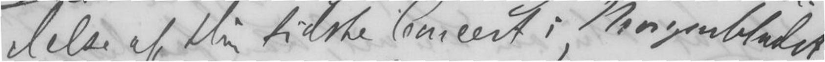delse af Din sidste Concert i Morgenbladet,
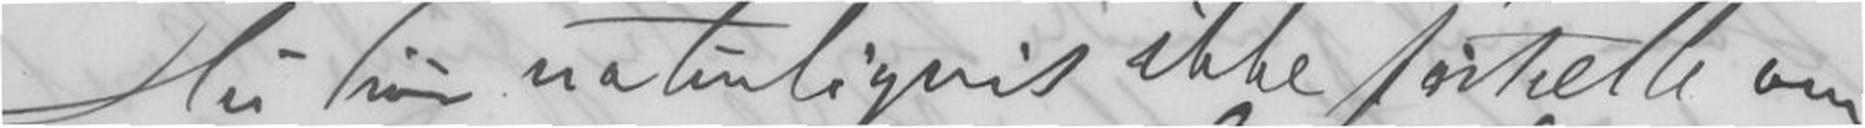Du bør naturligvis ikke fortælle om
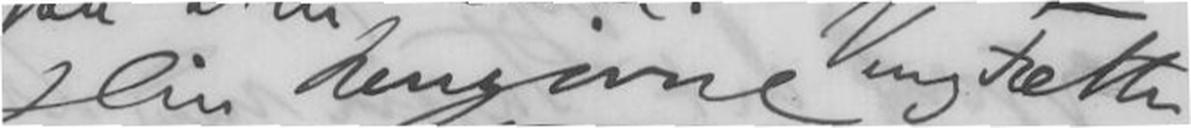Din hengivne Ven og Fætter
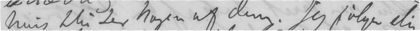hvis Du ser Nogen af dem. Jeg følger Dig
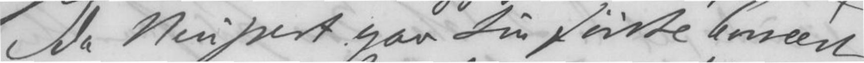Da Neupert gav sin første Concert,
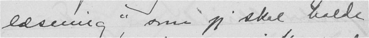læsning", som jeg skal holde
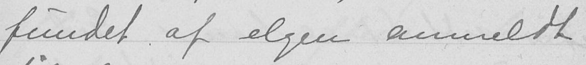fundet af elgen anmeldt
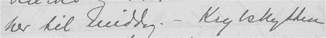her til middag - Krybskytten
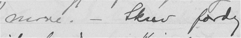mave. - Skrev ferdig
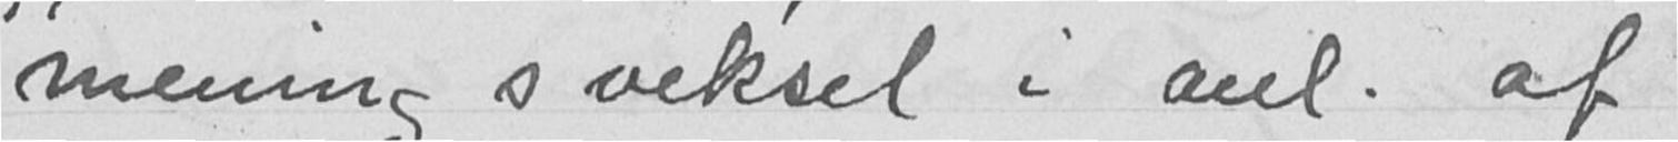meningsveksel i anl. af
